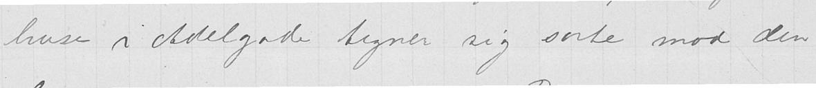huse i Adelgade tegner sig sorte mod den
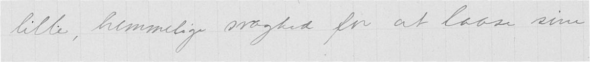lille, hemmelige svaghed for at laase sine
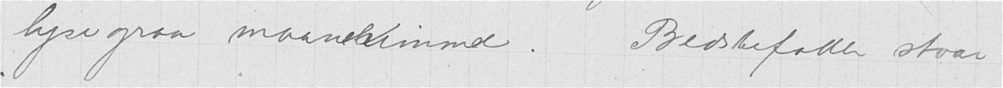lysegraa maanehimmel. Bedstefader staar
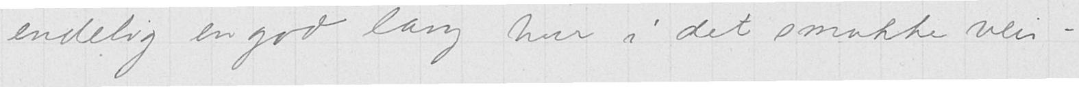endelig en god lang tur i det smukke veir -"
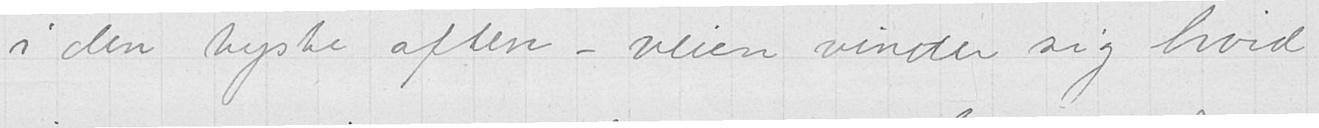i den kyske aften – veien vinder sig hvid
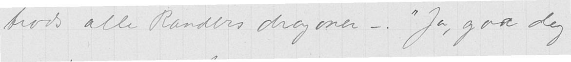trods alle Randers dragoner -. "Ja, gaa dog
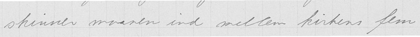skinner maanen ind mellem kirkens fem
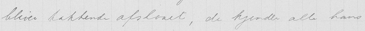bliver takkende afslaaet, de kjender alle hans
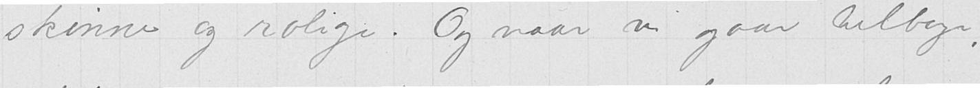skønne og rolige. Og naar vi gaar tilbage,
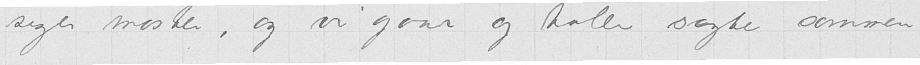siger moster, og vi gaar og taler sagte sammen
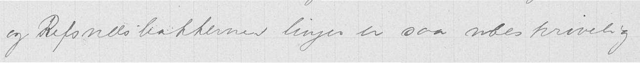og Refsnæsbakkernes linjer er saa ubeskrivelig
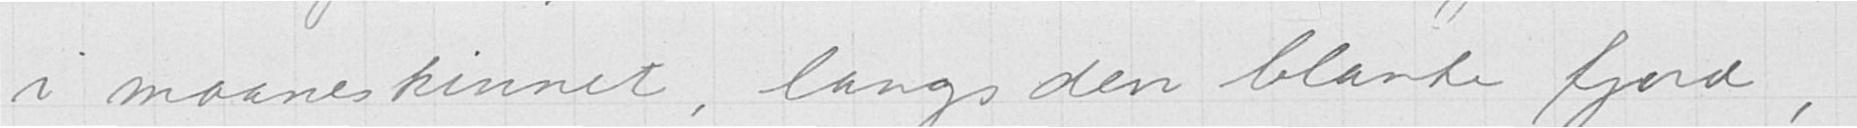i maaneskinnet, langs den blanke fjord,
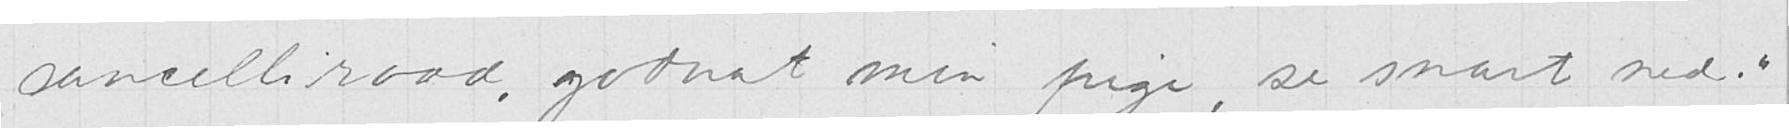cancelliraad, godnat min pige, se snart ned."
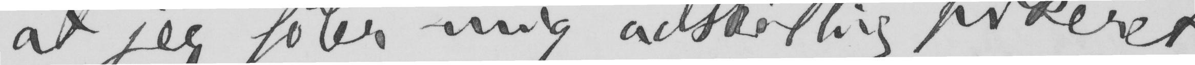at jeg føler mig adksillig pikeret
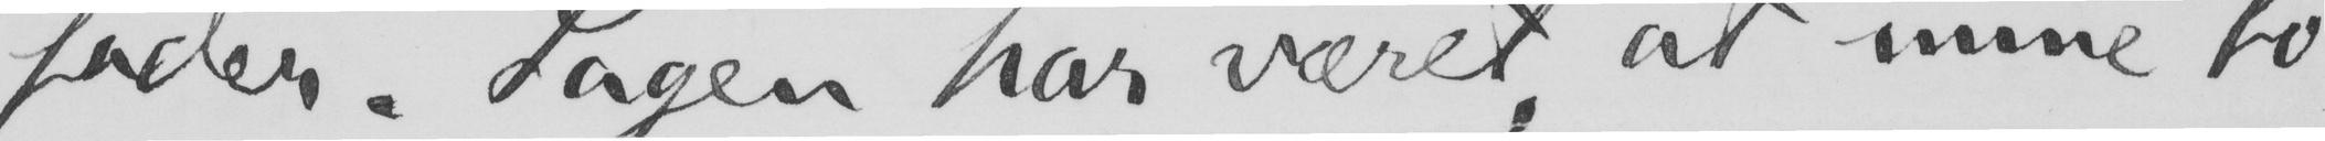fader. Sagen har været, at mine to
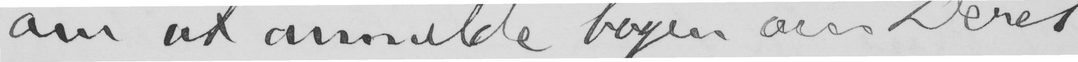om at anmelde bogen om Deres
